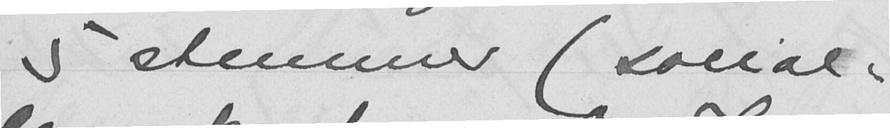5 stemmer (social-
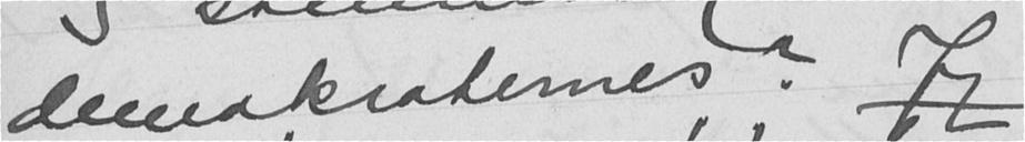demokraternes? Jeg
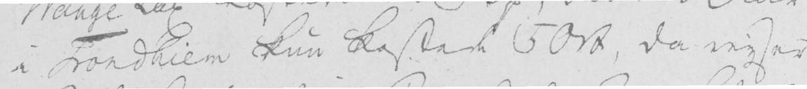i Trondhiem kun kostede 50 rd, da reyser
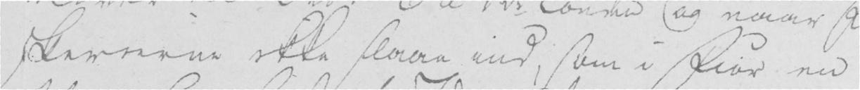skerierne ikke slaae ind, som i Fior en
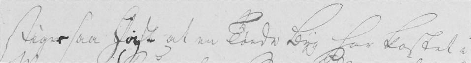stiger saa høyt at en Tønde Byg har kostet i
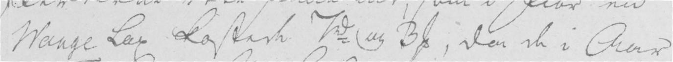Wange Lax kostede 7 rd og 3 s, da de i Aar
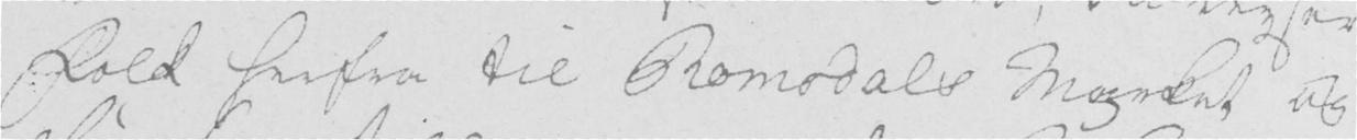Folk herfra til Romsdals Market og
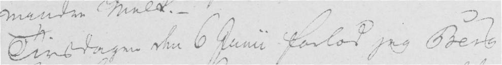Tirsdagen den 6 Junii forlod jeg Berg
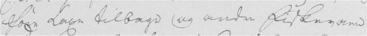føre Laxe tilbage og andre Fiskevand
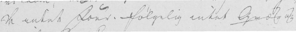de intet Foer, følgelig intet Qvæg og
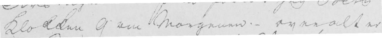Klokken 9 om Morgenen. - overalt er
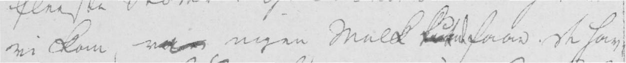vi kom, var ingen Melk  at faae. De hav-
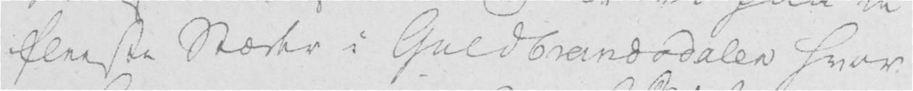fleeste Stæder i Guldbrandsdalen hvor
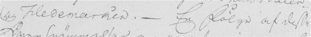og Hedemarken. - En Følge af disse
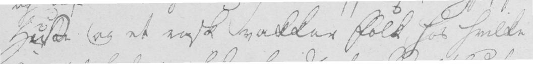Huse og et rask vakker Folk hos hvilke
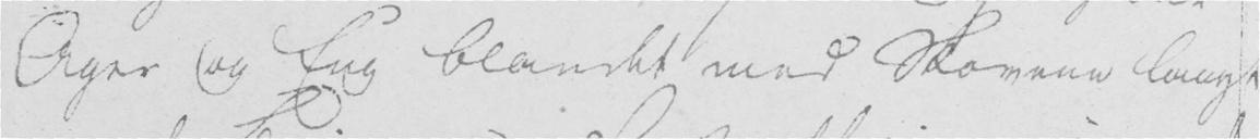Ager og Eng blandet med Skovene langt
